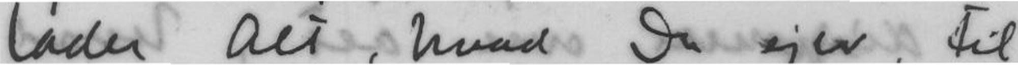lader Alt, hvad Du ejer, til
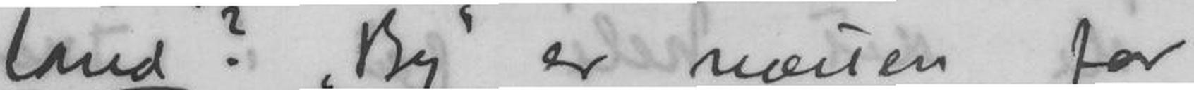land? By er næsten for
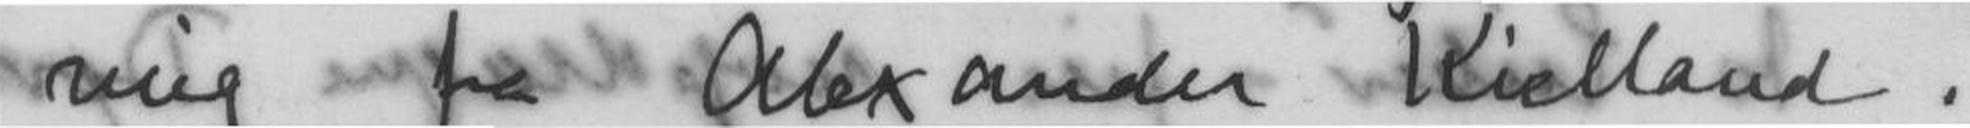ring fra Alexander Kielland.
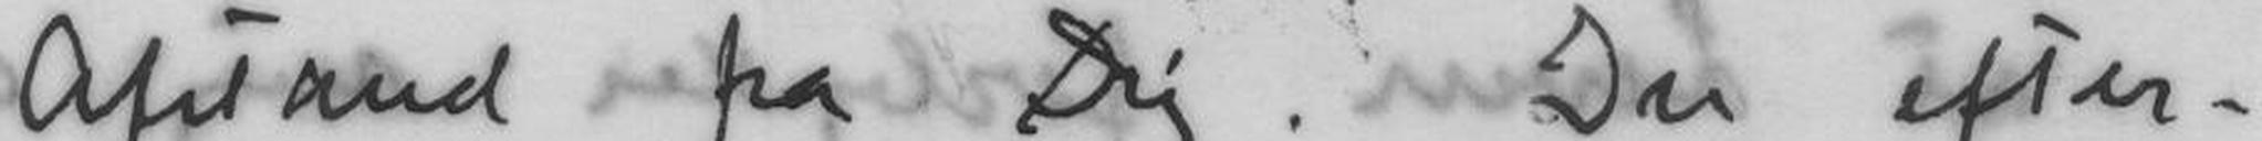Afstand fra Dig. Du efter-
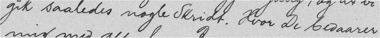gik saaledes nogle Skridt. Hvor De bedaarer
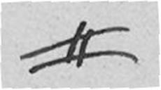#
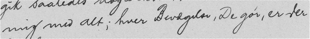mig med alt; hver Bevægelse, De gør, er der
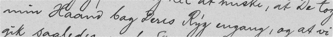min Haand bag Deres Ryg engang, og at vi
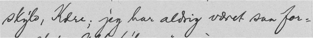skyld, Kære; jeg har aldrig været saa for-
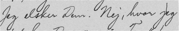Jeg elsker Dem. Nej, hvor jeg
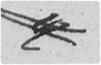#
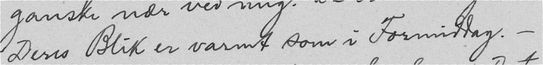Deres Blik er varmt som i Formiddag. -
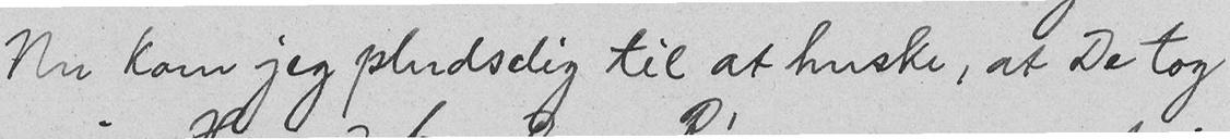Nu kom jeg pludselig til at huske, at De tog
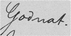Godnat.
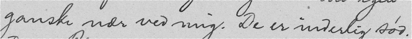ganske nær ved mig. De er inderlig sød.
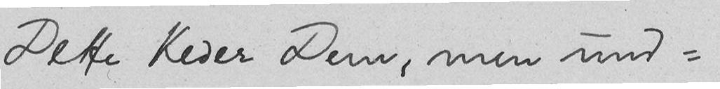Dette keder Dem, men und-
